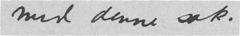med denne sak.
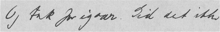Og tak for igaar. Gid det ikke
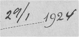29/1 1924
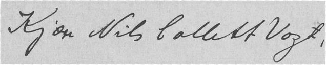Kjære Nils Collett Vogt,
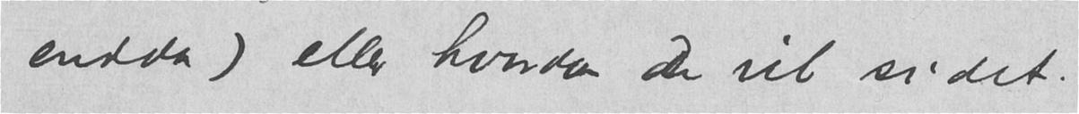endda) eller hvordan de vil si det.
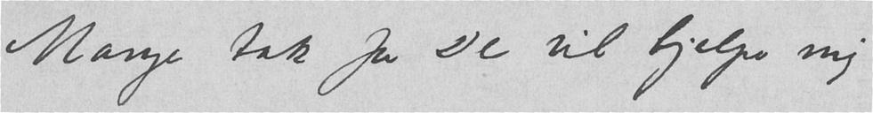Mange tak for De vil hjelpe mig
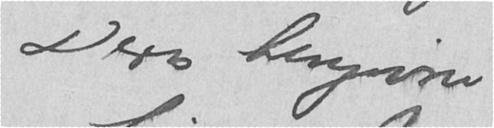Deres hengivne
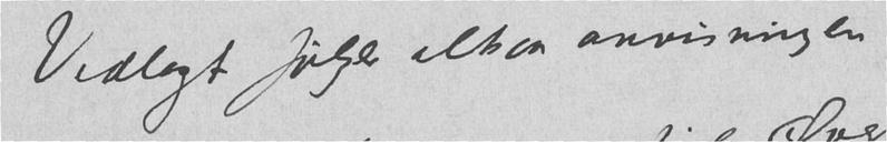Vedlagt følger altsaa anvisningen
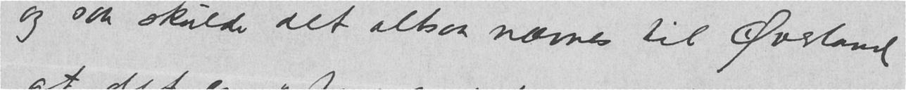og saa skulde det altsaa nævnes til Øverland
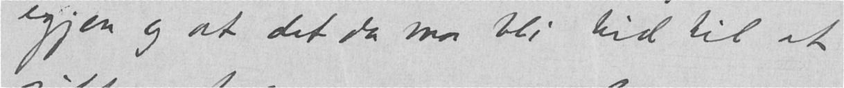igjen og at det da maa bli tid til at
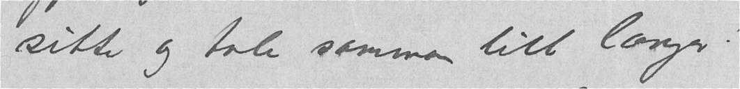sitte og tale sammen litt længer!
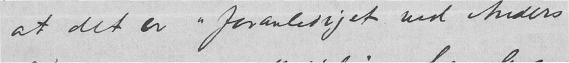at det er «foranlediget ved Anders
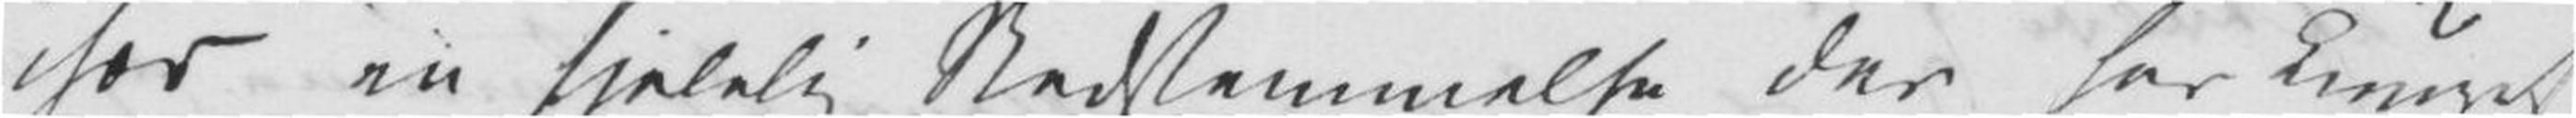især en sjelelig Nedstemmelse der har knuget
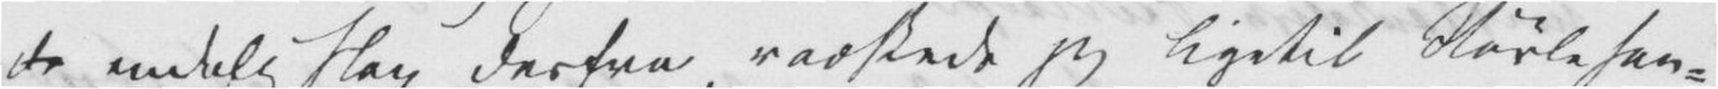d<gap /></del> endelig slap derfra, radskede jeg ligetil Støvlehan¬
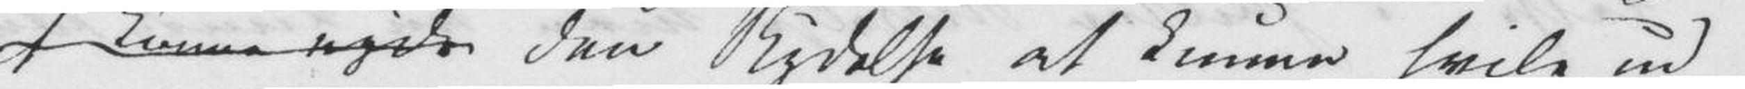at kunne nyde</del> den Nydelse at kunne hvile ud
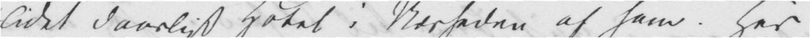lidet daarligt Hotel i Nærheden af ham. Her
